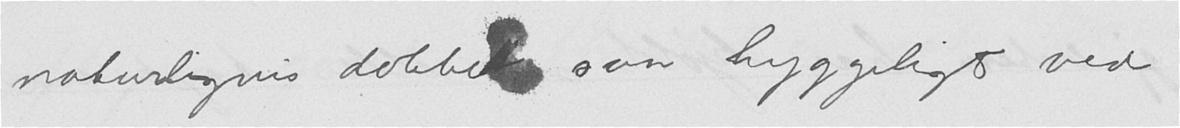naturligvis dobbelt saa hyggeligt ved
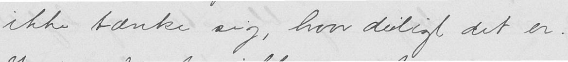ikke tænke sig, hvor deiligt det er.
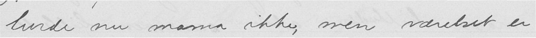turde nu mamma ikke, men værelset er
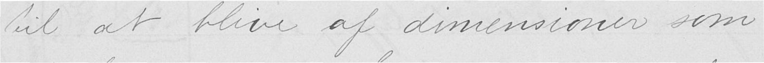til at blive af dimensioner som
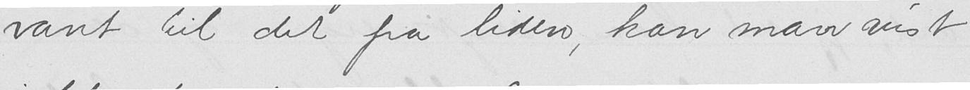vant til det fra liden, kan man vist
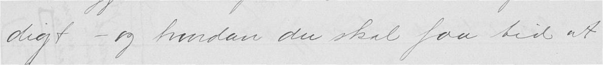digt - og hvordan du skal faa tid at
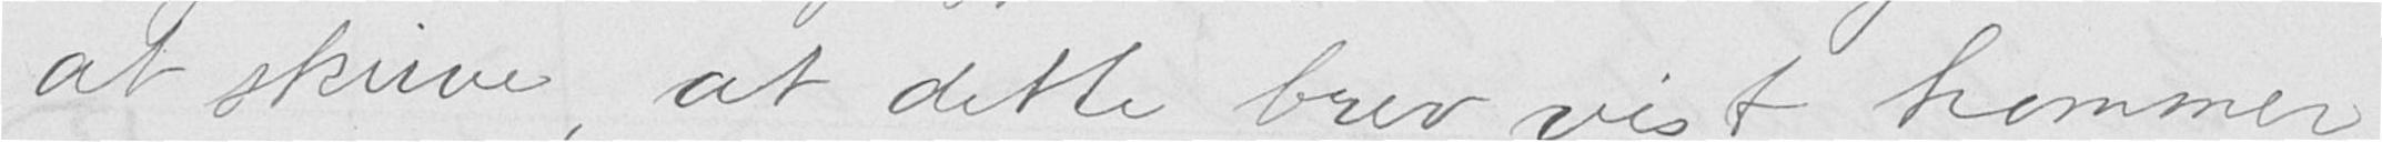at skrive, at dette brev vist kommer
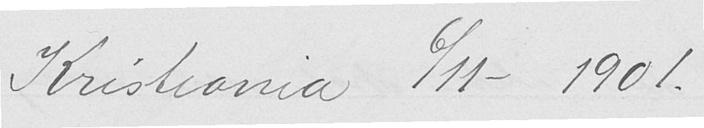Kristiania 6/11-1901.
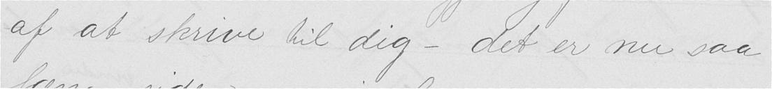af at skrive til dig - det er nu saa
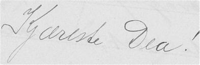Kjæreste Dea!
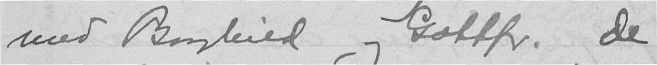med Borghild og Gottfr. de
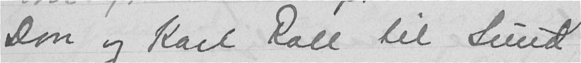Don og Karl Roll til Sund
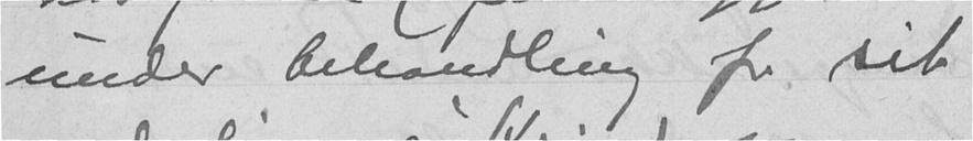under behandling for sit
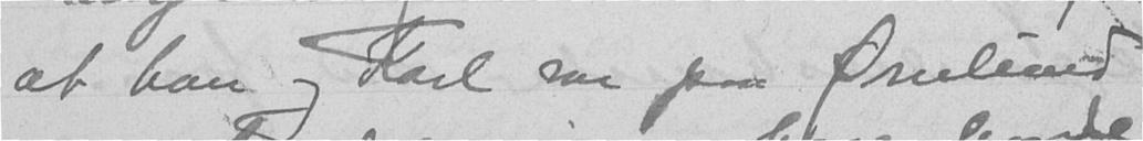at han og Karl var paa Ørnelund
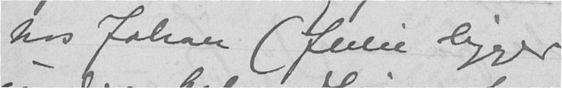hos Johan (Julie ligger
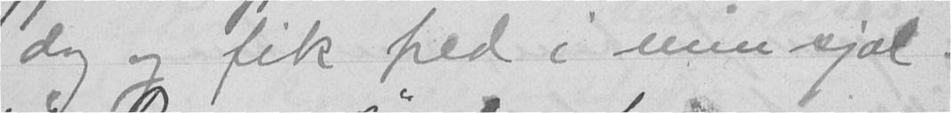dag og fik fred i min sjæl.
